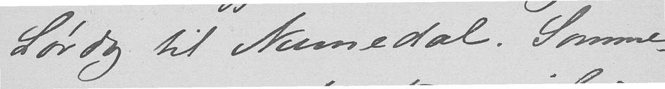Lørdag til Numedal. Somme-
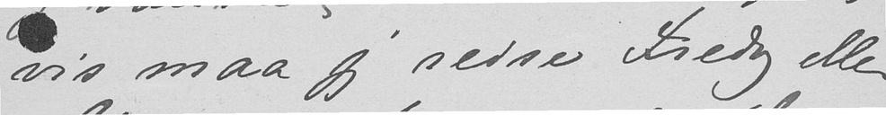vis maa jeg reise Fredag eller
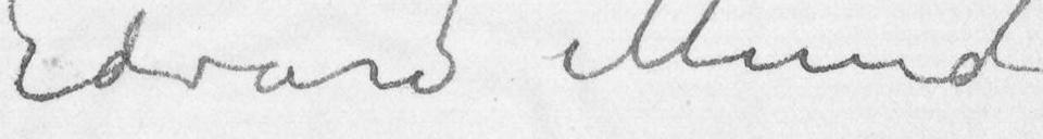Edvard Munch
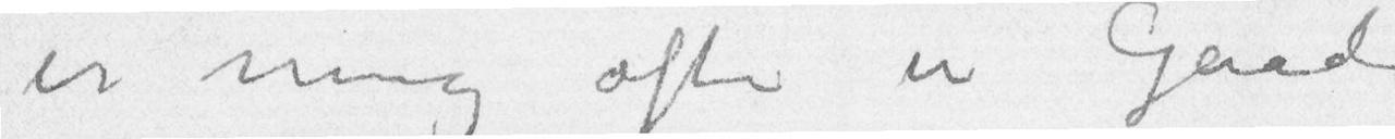er mig ofte en Gaade
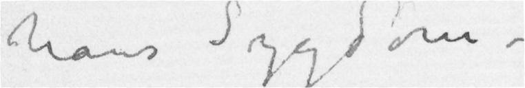hans Sygdom –
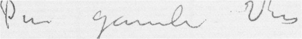Din gamle Ven
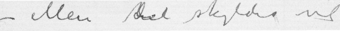– Men det skyldes vel
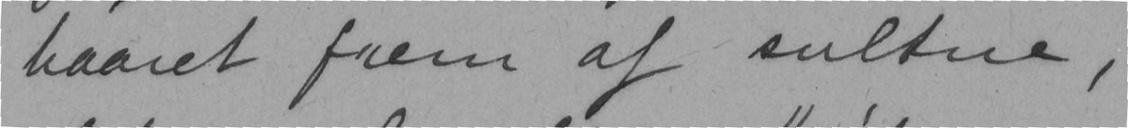baaret frem af sultne,
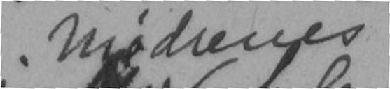mødrenes
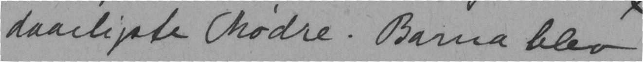daarligste Mødre. Barna blev
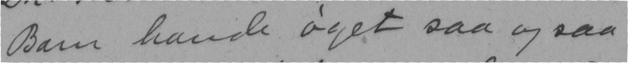Barn havde øget saa og saa
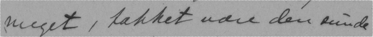meget, takket være den sunde
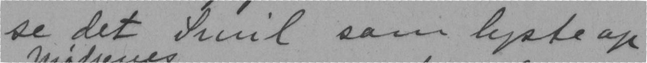se det Smil som lyste op
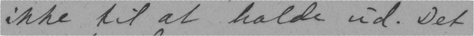ikke til at holde ud. Det
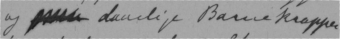og  daarlige Barne kropper
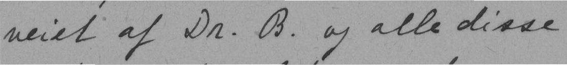veiet af Dr. B. og alle disse
